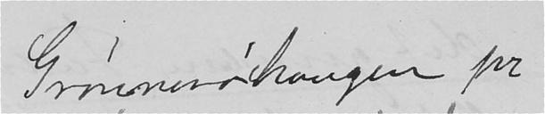Grønnesørhaugen pr
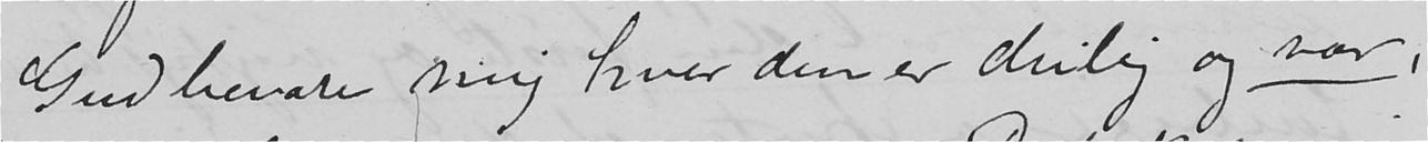Gudbevare mig hvor den er deilig og vær-
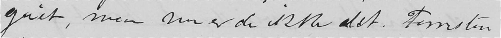gået, men nu er de ikke det. Forresten
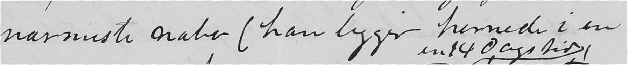nærmeste nabo (han ligger hernede i en
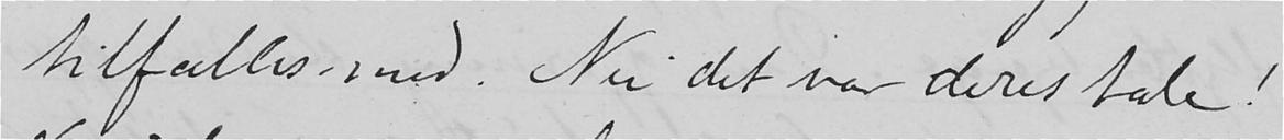tilfælles med. Nu det var deres tale!
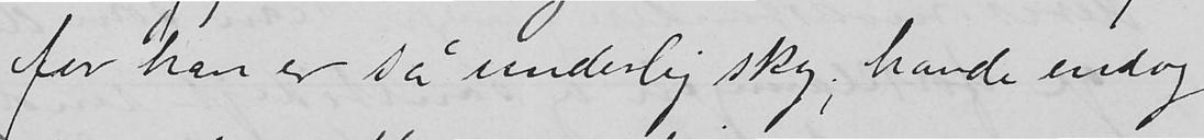for han er så underlig sky, havde endog
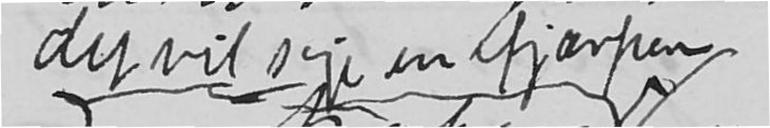det vil sige en fjærpen
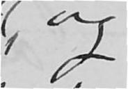, og
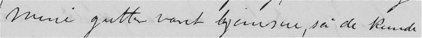mine gutter været hjemme, så de kunde
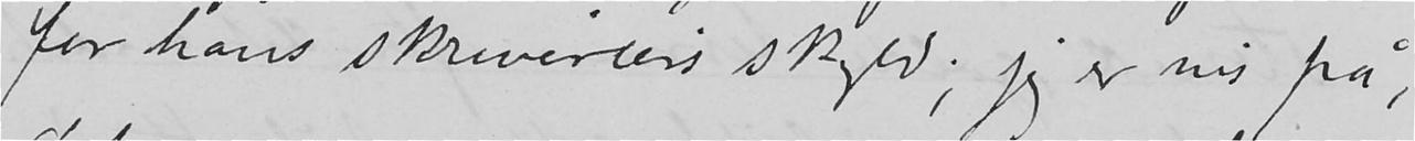for hans skriveriers skyld; jeg er vis på,
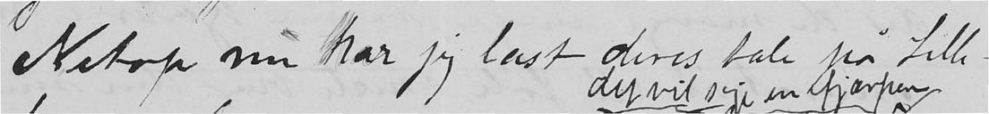Netop nu har jeg læst deres tale på Lille-
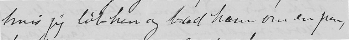hvis jeg løb hen og bad ham om en pen,
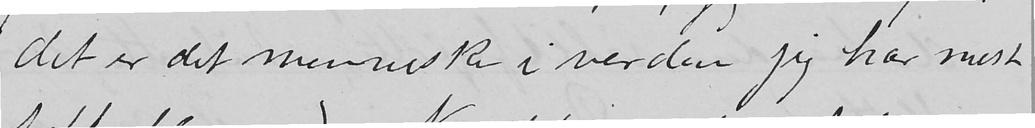det er det menneske i verden jeg har mest
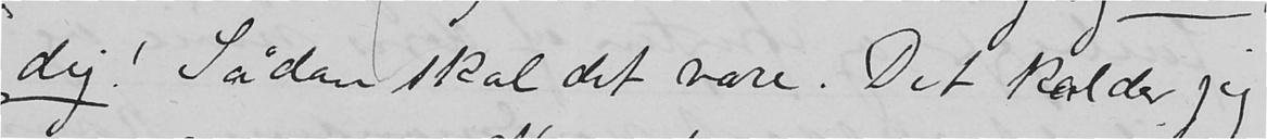dig! Sådan skal det være. Det kalder jeg
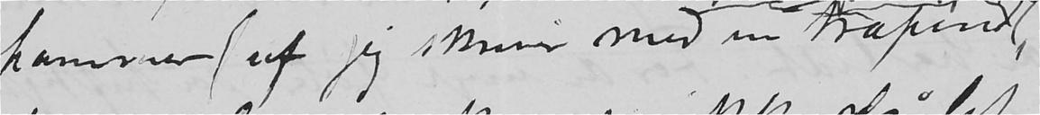hammer (uf jeg skriver med en træpind,
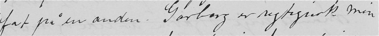fat på en anden. Garborg er rigtignok min
